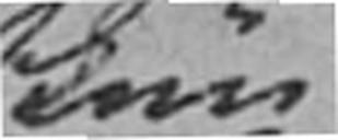kun
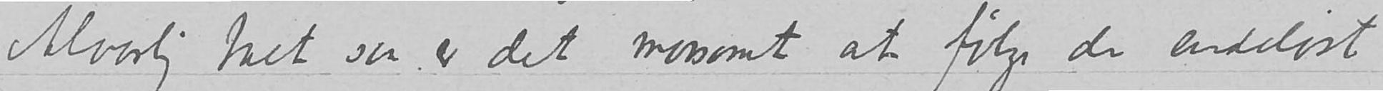Alvorlig talt saa er det morsomt at følge de endeløst
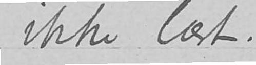ikke læst.
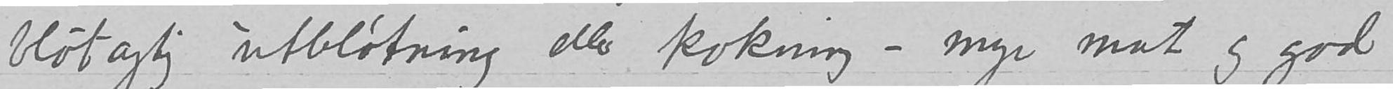bløtagtig utbløtning eller kokning - mye mat og god
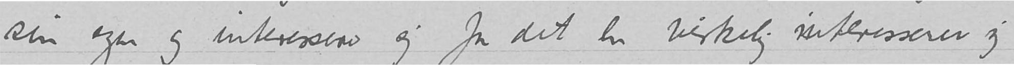sin egen og interessere sig for det en virkelig interesserer sig
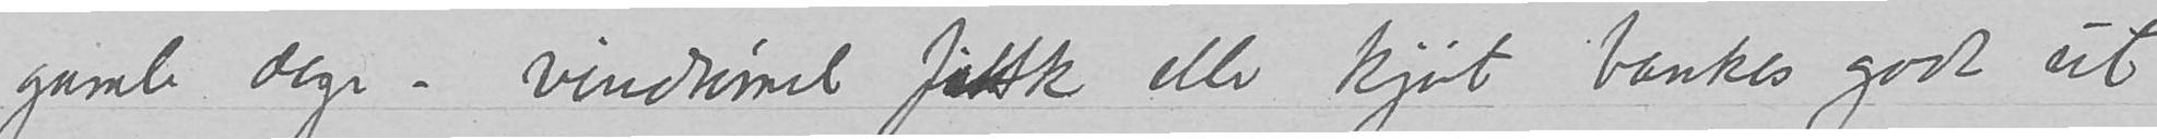gamle dager - vindtørret fisk eller kjøt bankes godt ut
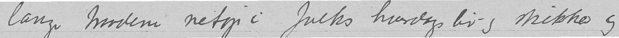lange traadene netop i folks hverdagsliv - og skikker og
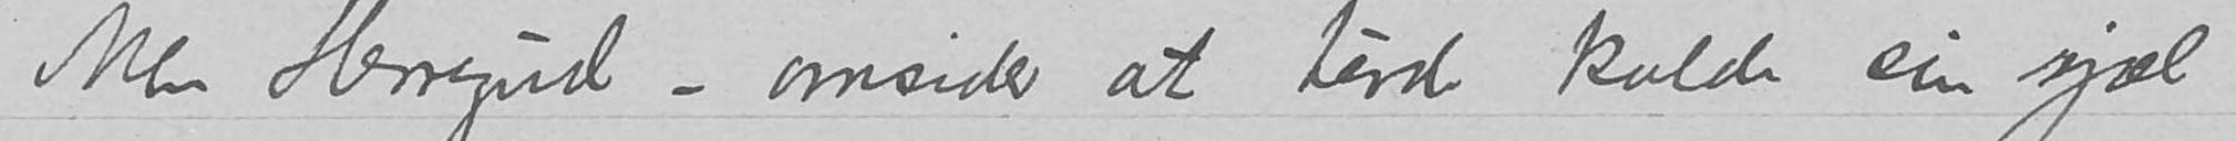Men Herregud - omsider at burde kalde sin sjæl
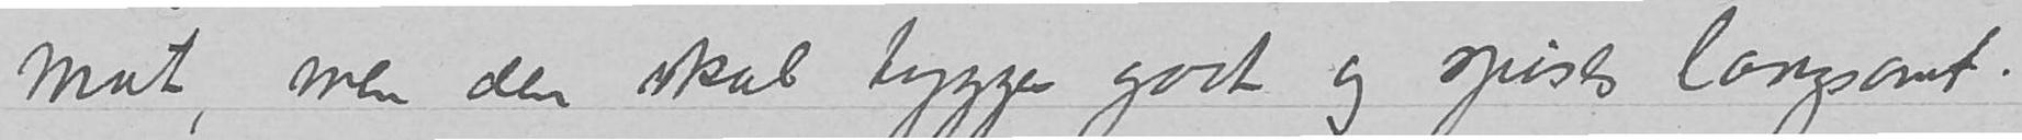mat, men den skal tygges godt og spises langsomt.
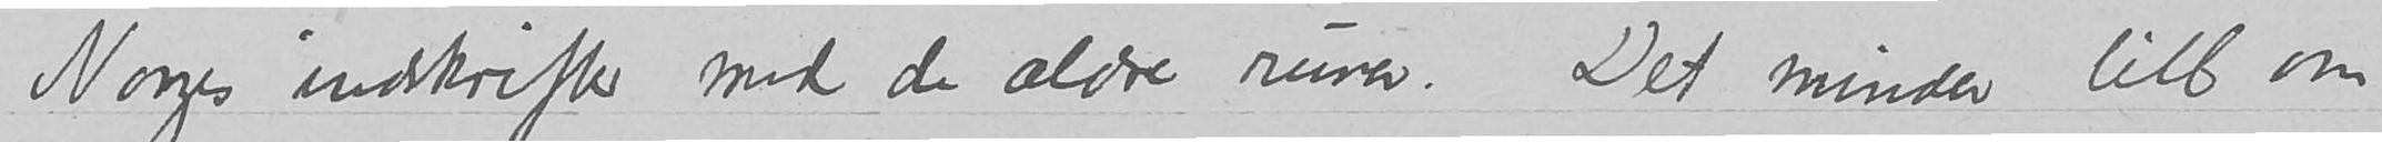Norges indskrifter med de ældre runer. Det minder litt om
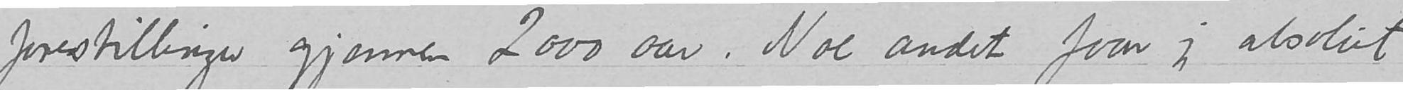forestillinger gjenneom 2000 aar.  Noe andet faar jeg absolut
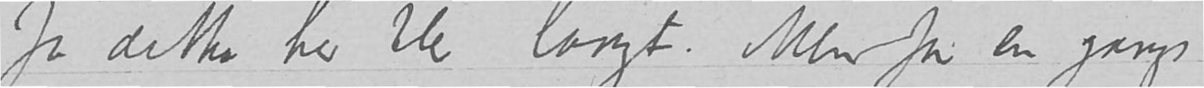Ja dette her blev langt.   Men for en gangs
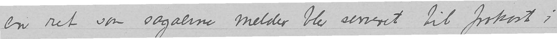en ret som sagaerne melder blev serveret til frokost i
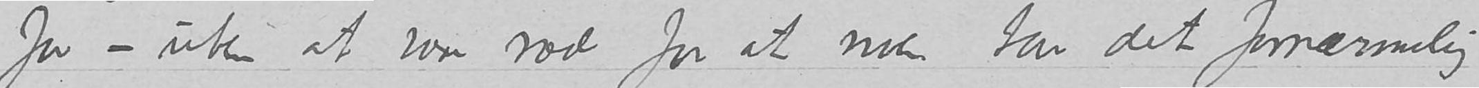for - uten at være ræd for at noen tar det fornærmelig
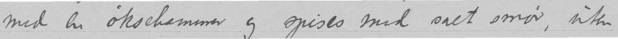med en øksehammer og spises med salt smør, uten
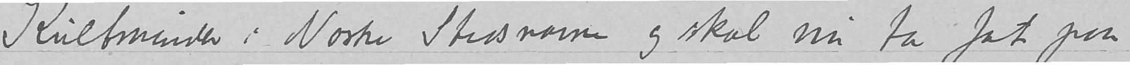Kulturminder i Norske Stedsnavn og skal nu ta fat paa
